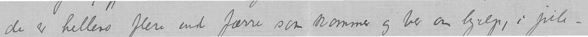de er hellere flere end færre som kommer og ber om hjelp, i jule-
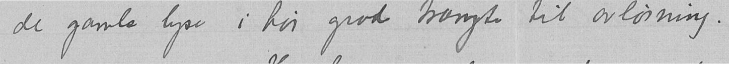de gamle lyse i høi grad trængte til avløsning.
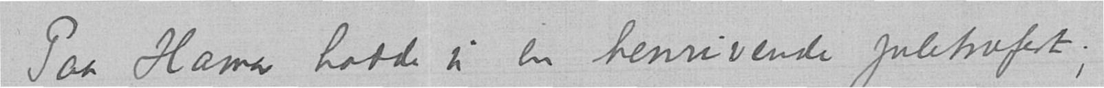Paa Hamar hadde vi en henrivende juletræfest;
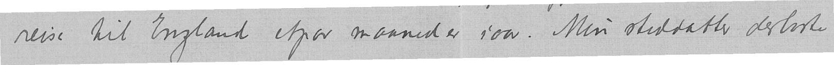reise til England etpar maaneder iaar. Min steddatter derborte
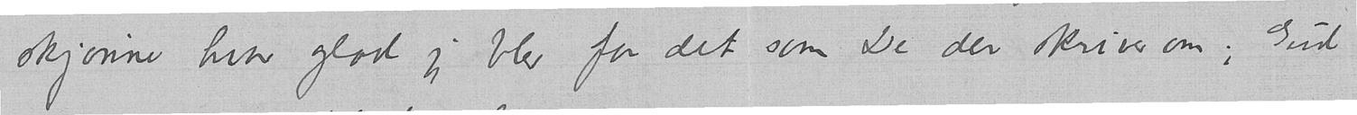skjønne hvor glad jeg blev for det som De der skriver om; Gud
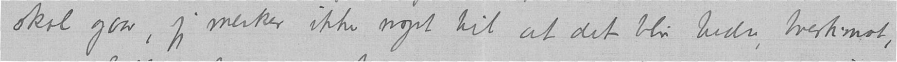skal gaa, jeg merker ikke noget til at det blir bedre, tvertimot,
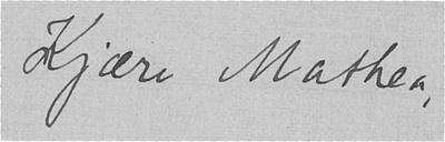Kjære Mathea,
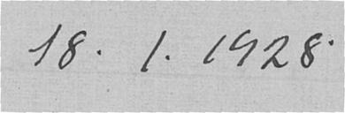18.1.1928.
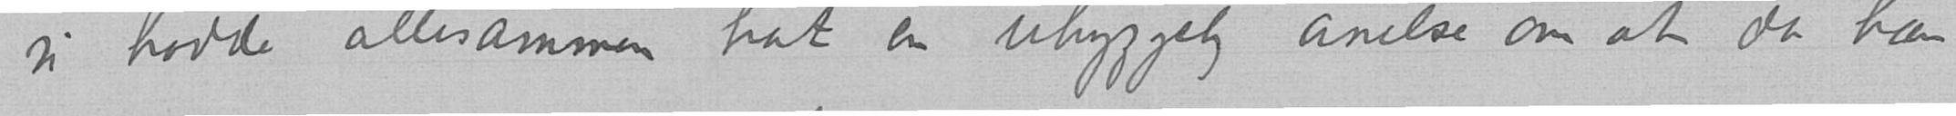vi hadde allesammen hat en uhyggelig anelse om at da han
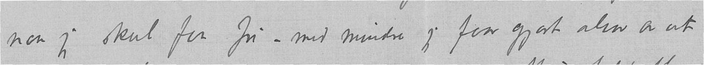naar jeg skal faa fri – med mindre jeg faar gjort alvor av at
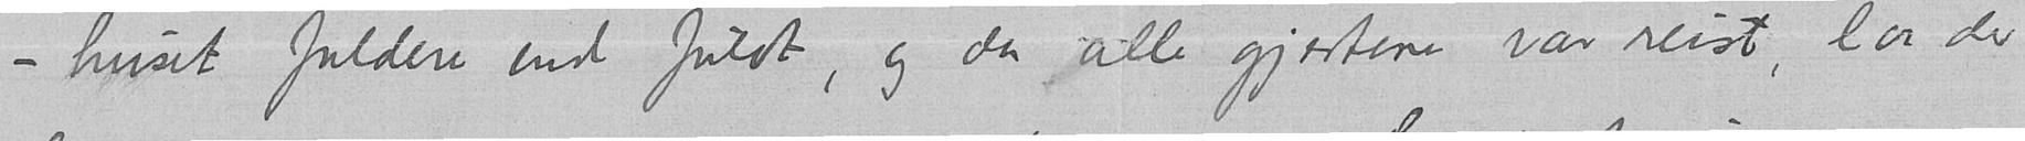– huset fuldere end fuldt, og da alle gjestene var reist, laa der
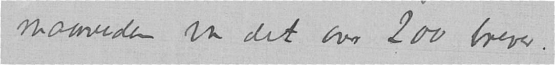maaneden var det over 200 brever.
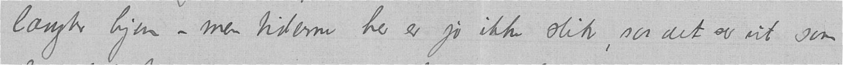længter hjem – men tiderne her er jo ikke slik, saa det ser ut som
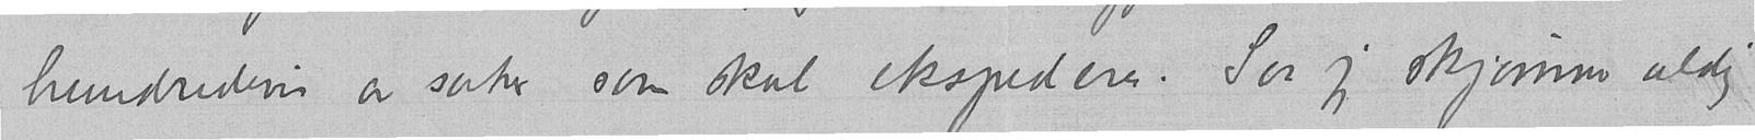hundredevis av saker som skal ekspederer. Saa jeg skjønner aldrig
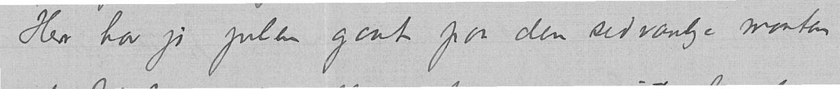Her har jo julen gaat paa den sedvanlige maaten
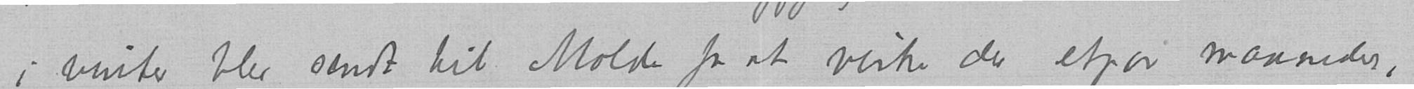i vinter blev sendt til Molde for at virke der etpar maaneder,
